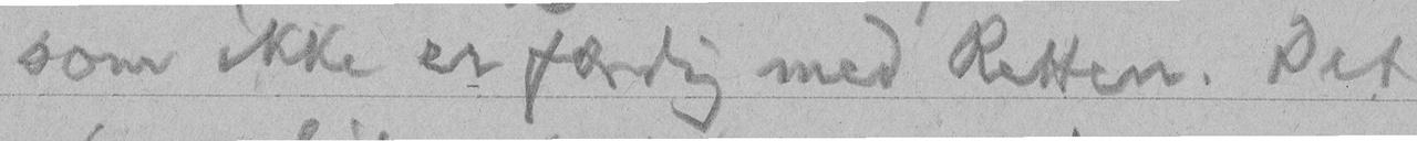som ikke er færdig med Retten. Det
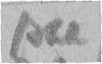saa
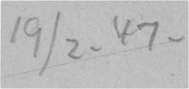19/2. 47.
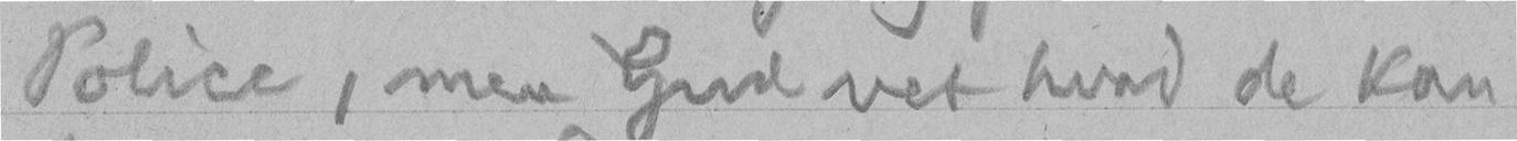Police, men Gud vet hvad de kan
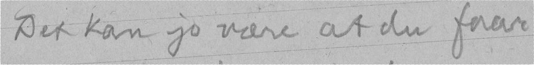Det kan jo være at du faar
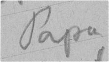Papa
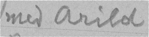med Arild
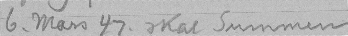6. Mars 47. skal Summen
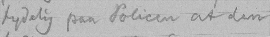tydelig paa Policen at den
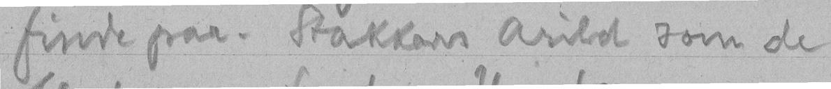finde paa. Stakkars Arild som de
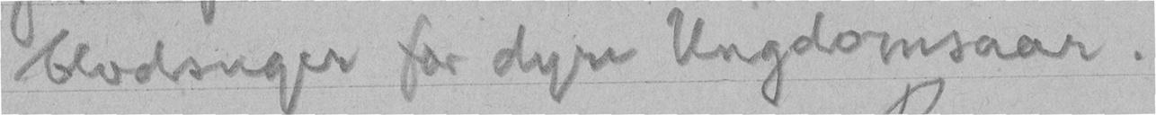blodsuger for dyre Ungdomsaar.
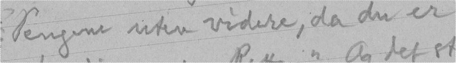Pengene uten videre, da du er
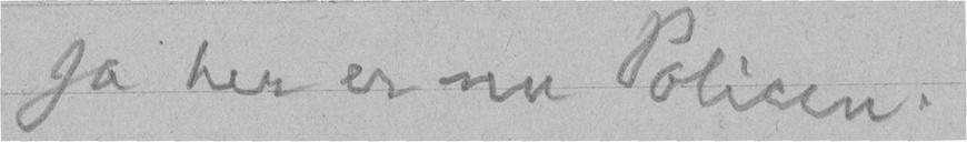Ja her er nu Policen.
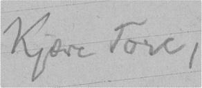Kjære Tore,
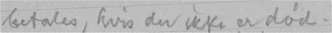betales, hvis du ikke er død.
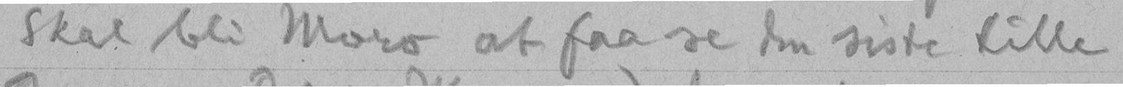Skal bli Moro at faa se den siste lille
